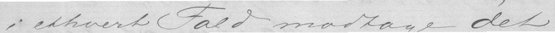i ethvert Fald modtage det
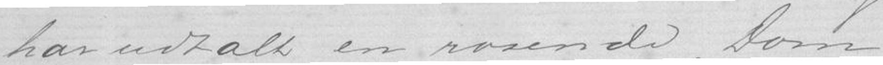har udtalt en rosende Dom
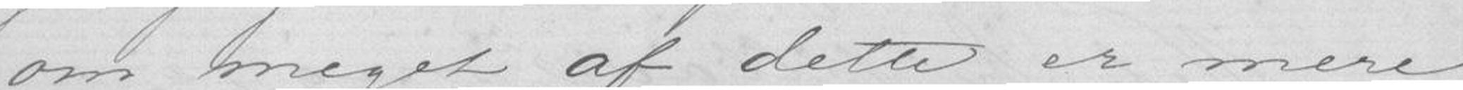om meget af dette er mere
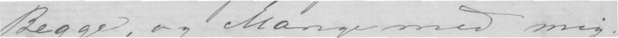Begge, og Mange med mig;
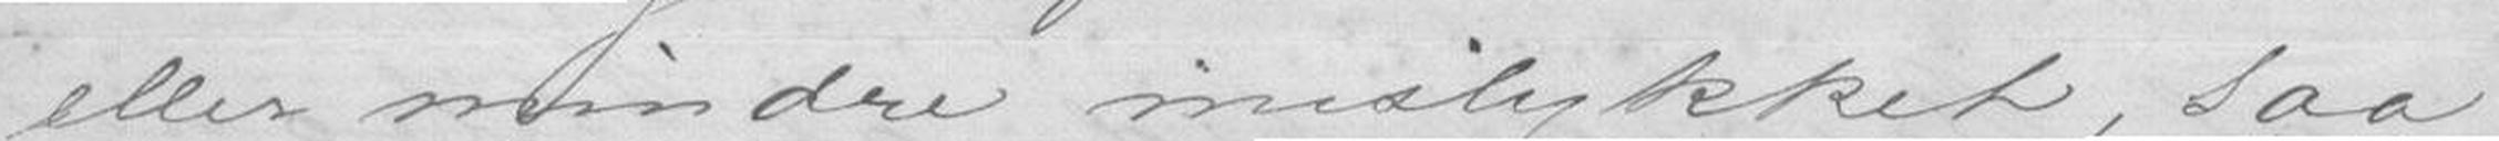eller mindre mislykket, saa
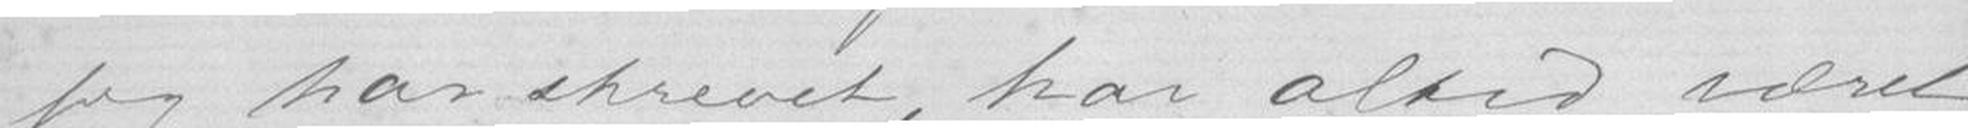jeg har skrevet, har altid været
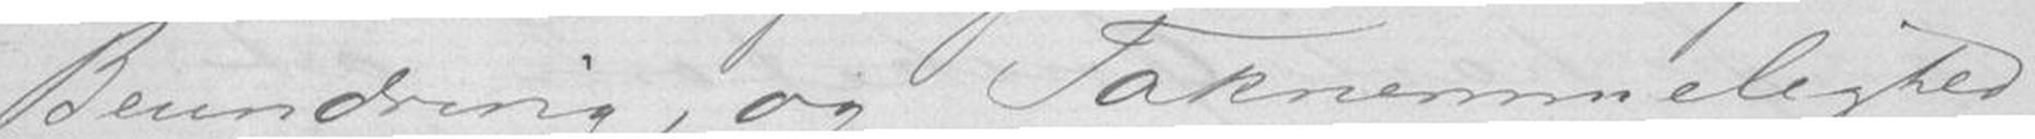Beundring, og Taknemmelighet
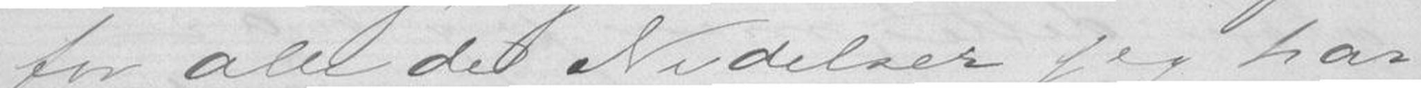for alle de Nydelser jeg har
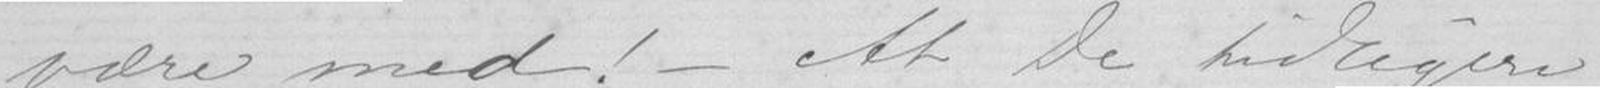være med! - At De tidligere
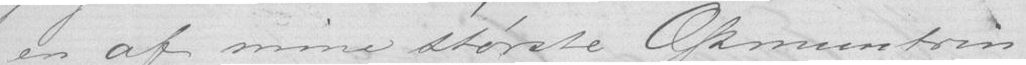en af mine største Opmuntrin
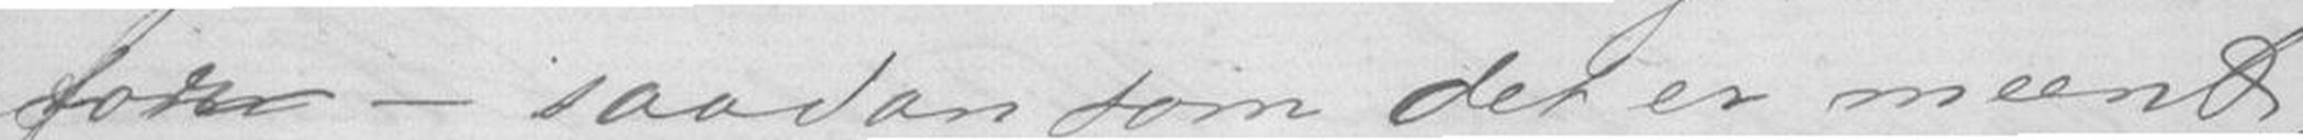for - saadan som det er meent, -
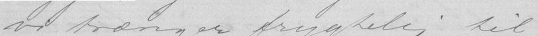vi trænger frygtelig til
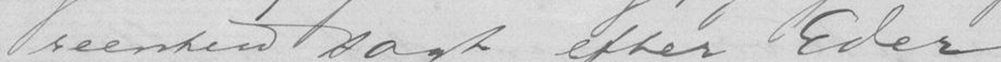reentud sagt, efter Eder
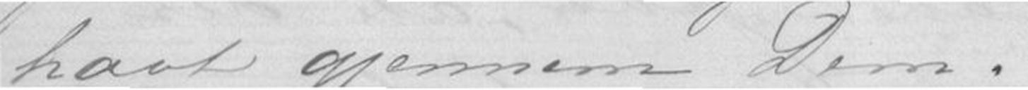havt gjennem Dem.
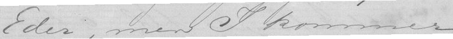Eder, men I kommer
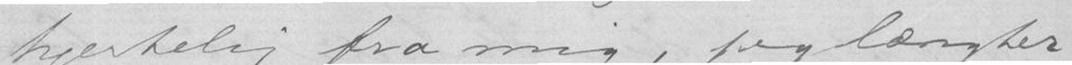hjertelig fra mig, jeg længter
